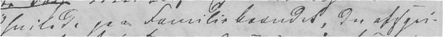hvilede paa Familiebaandet, der afspei-
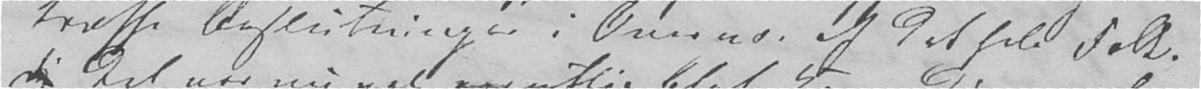træffe Belutninger i Census, af det hele Folk.
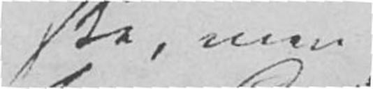ske, men
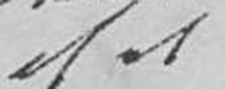af et
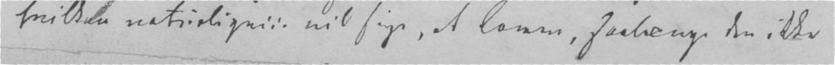hvilken naturligviis vil sige, at Loven, saalænge den ikke
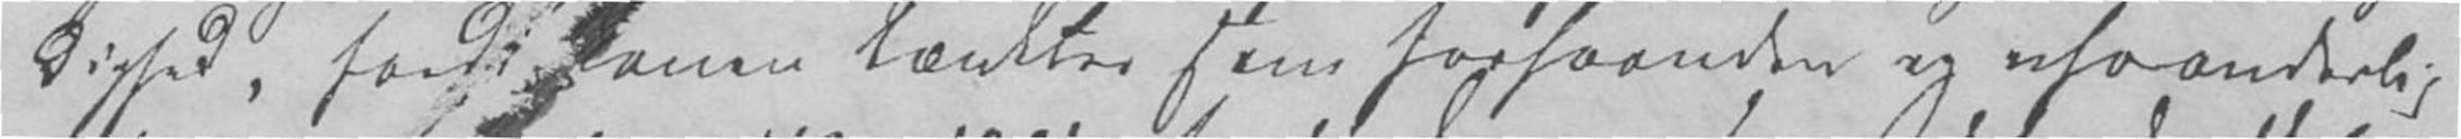dighed, fordi Loven tænktes som forhaanden og uforanderlig
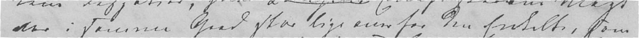var i Samme Grad stor lige overfor den Enkelte, som
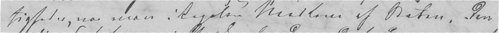tigheder, var man i Regelen Medlem af Staten. Den
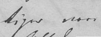Riger vare
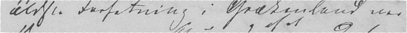ældste Forfatning i Grækenland var
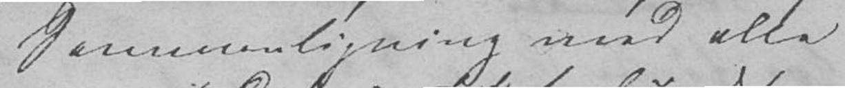Sammenligning med alle
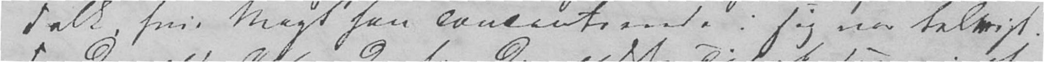Folk, hvis Magt han concentrerede i sig var taltrigt.
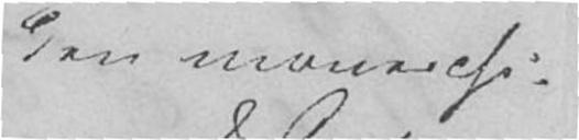den monarchi-
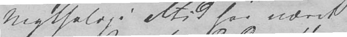Mythologi altid har været
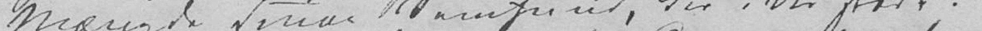Mængde smaae Samfund, der ikke stode i
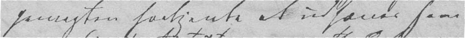gemagten fortjente at udhæves som
